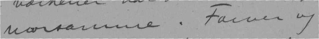morsomme. Farver og
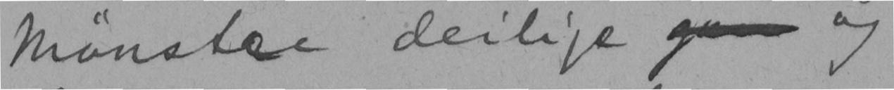Mønstre deilige  og
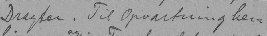Dragter. Til Opvartning her-
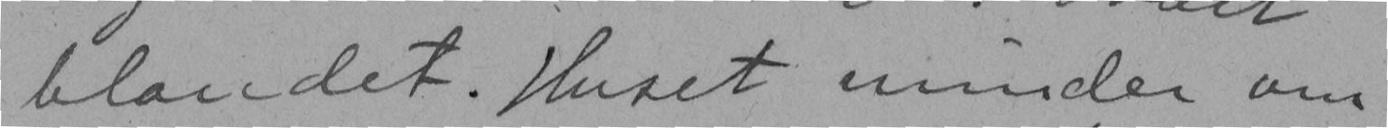blandet. Huset minder om
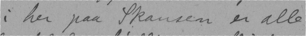i her paa Skansen er alle
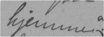hjemme
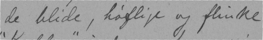de blide, høflige og flinke
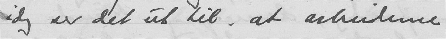idag ser det ut til, at arbeiderne
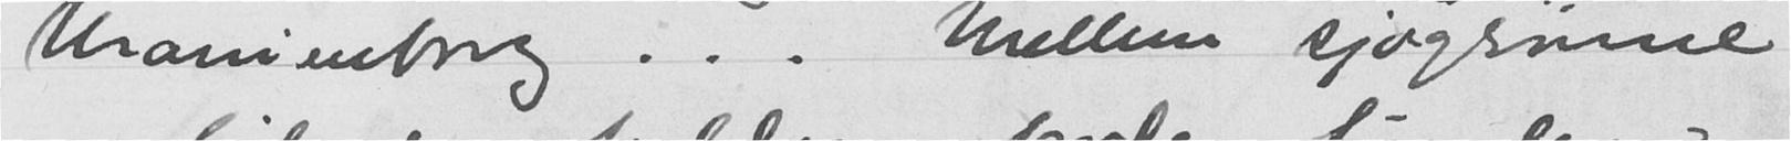Uranienborg... Mellem sjøgrønne
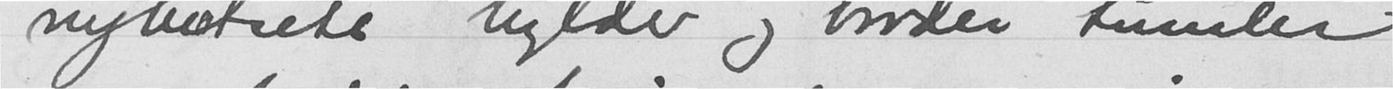nybitsete hylder og border tumler
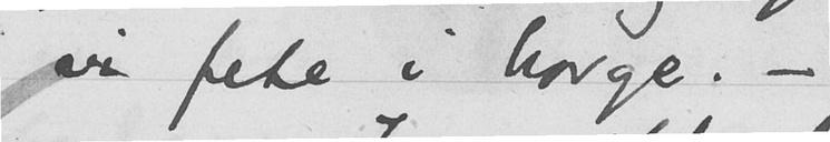vi fete i Norge. -
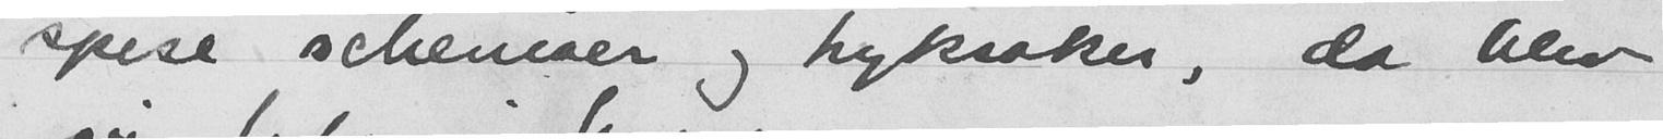spise schemaer og tryksaker, da blev
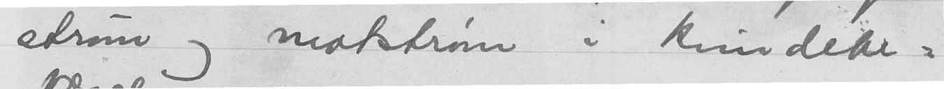strøm og motstrøm i kvindebe-
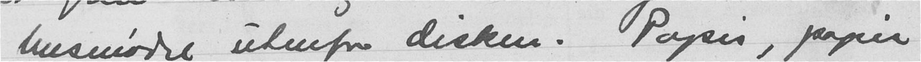husmødre utenfor disken. Papir, papir
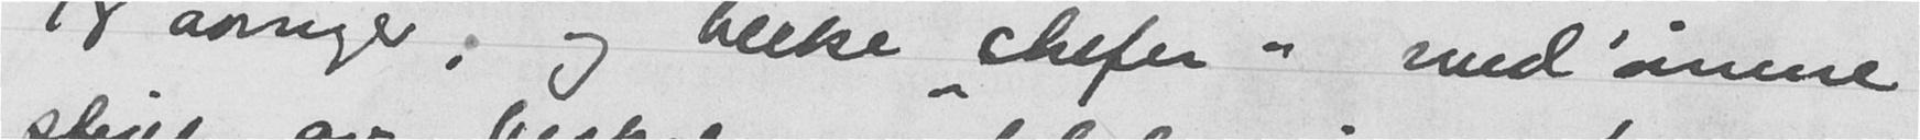18 aaringer, og belke "chefer" med øinene
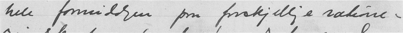hele formiddagen paa forskjellige ratione-
